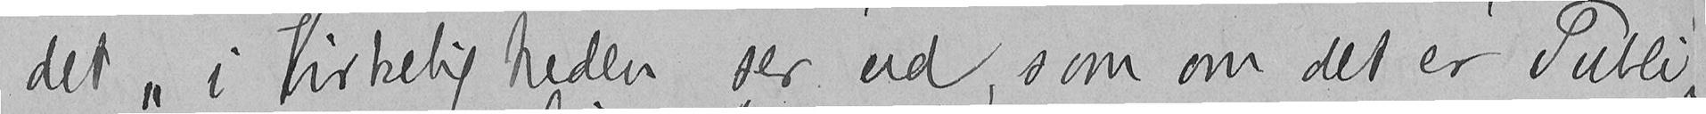det "i Virkeligheden ser ud, som om det er Publi-
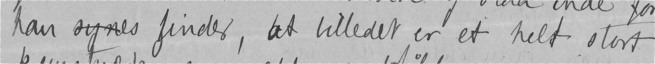han synes finder, at billedet er et helt stort
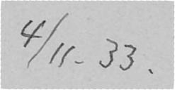4/11. 33.
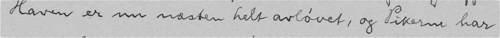Haven er nu næsten helt avløvet, og Pikerne har
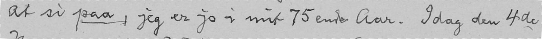at si paa, jeg er jo i mit 75 ende Aar. Idag den 4de
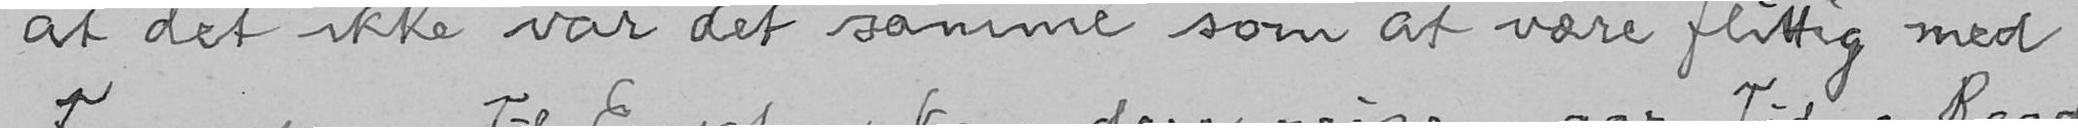at det ikke var det samme som at være flittig med
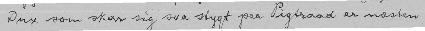Dux som skar sig saa stygt paa Pigtraad er næsten
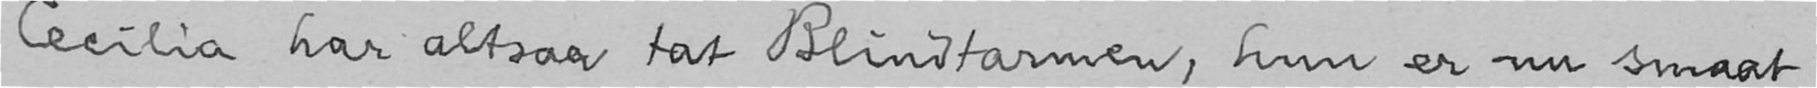Cecilia har altsaa tat Blindtarmen, hun er nu smaat
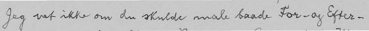Jeg vet ikke om du skulde male baade For- og Efter-
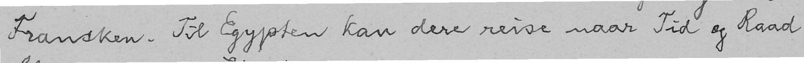Fransken. Til Egypten kan dere reise naar Tid og Raad
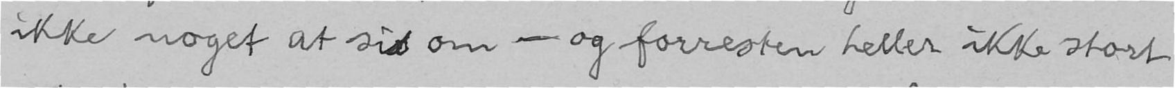ikke noget at sid om - og forresten heller ikke stort
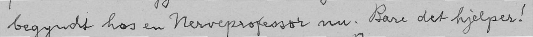begyndt hos en Nerveprofessor nu. Bare det hjelper!
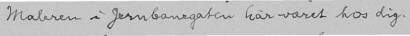Maleren i Jernbanegaten har været hos dig.
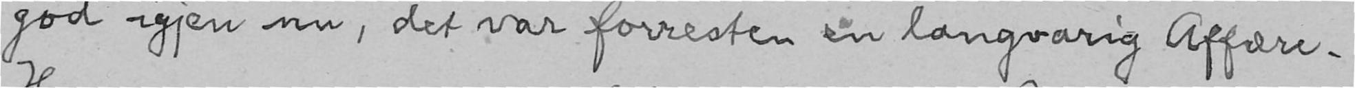god igjen nu, det var forresten en langvarig Affære.
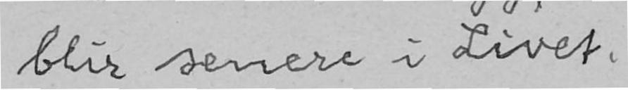blir senere i Livet.
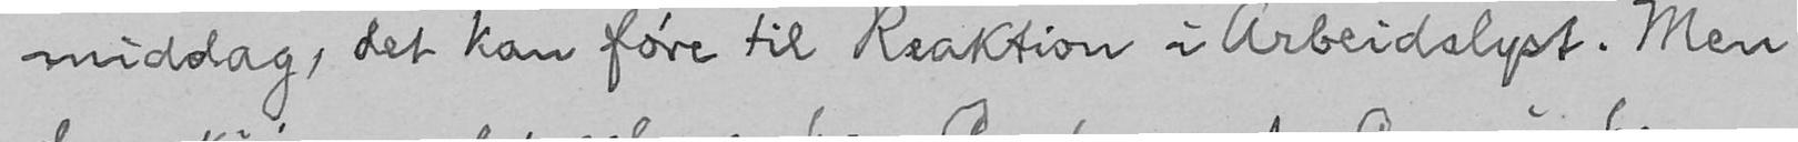middag, det kan føre til Reaktion i Arbeidslyst. Men
